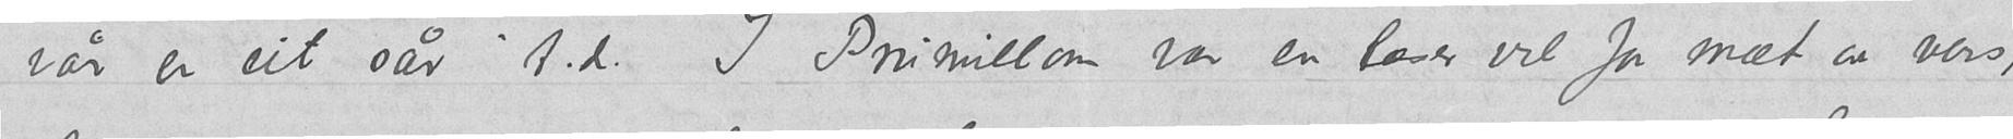vår er eit sår" t.d. I Brumillom var en læser vel for mæt av vers,
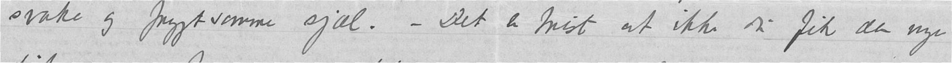svake og frygtsomme sjæl. – Det er trist at ikke du fik den nye
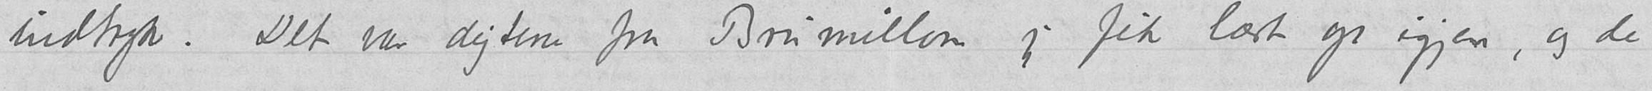indtryk. Det var digtene fra Brumillom jeg fik læst op igjen, og de
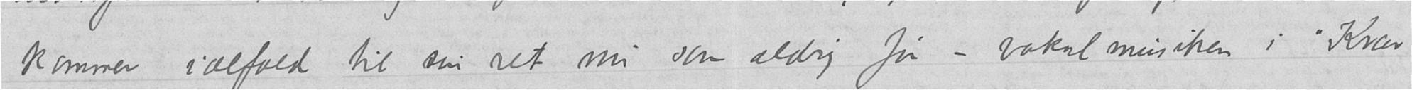kommer ialfald til sin ret nu som aldrig før – vokalmusiken i "Kvar
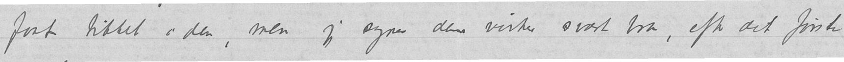faat tittet i den, men jeg synes den virker svært bra, efter det første
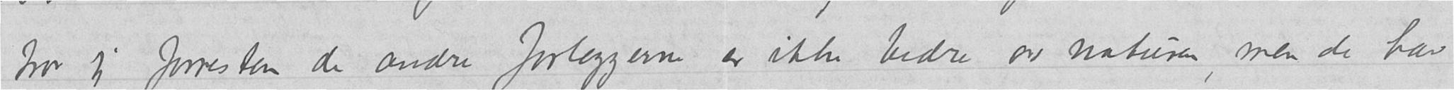tror jeg forresten de andre forlæggerne er ikke bedre av naturen, men de har
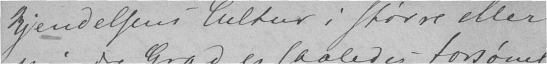kjendelsens Cultur i større eller
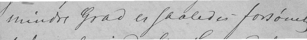mindre Grad er saaledes forsømt,
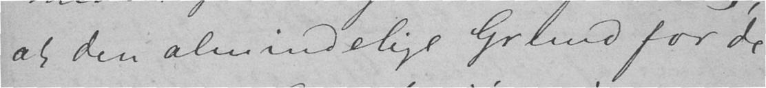at den almindelige Grund for de
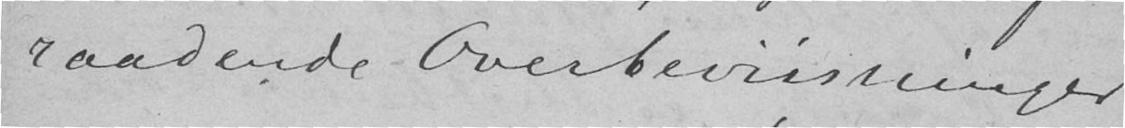raadende Overbeviisninger
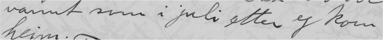varmt som i juli etter eg kom
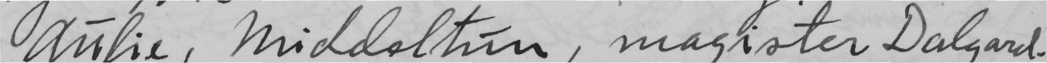Aulie, Middeltun, magister Dalgard.
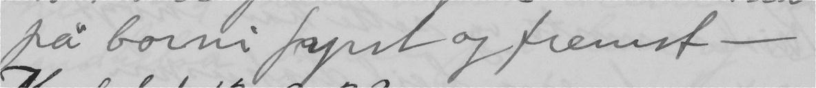på borni fyrst og fremst –
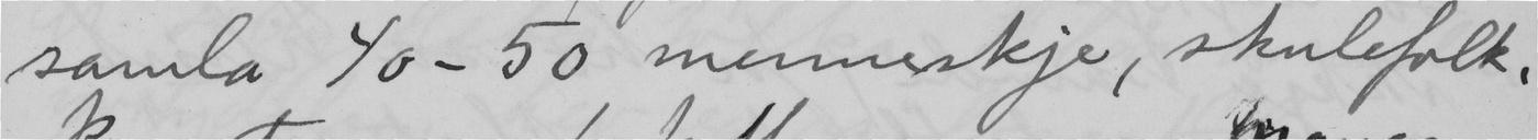samla 40-50 menneskje, skulefolk,
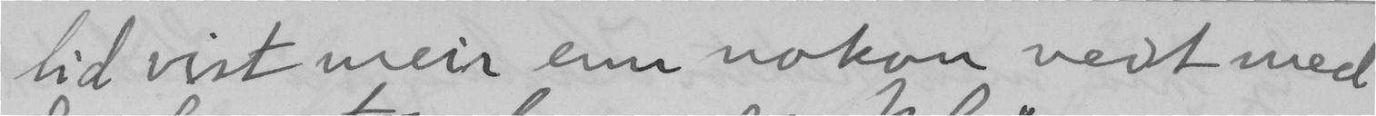lid vist meir enn nokon veit med
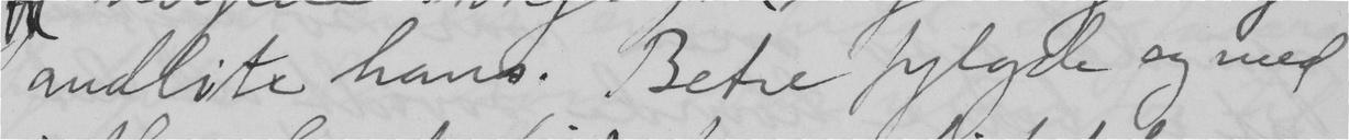andlite hans. Betre fylgde eg med
